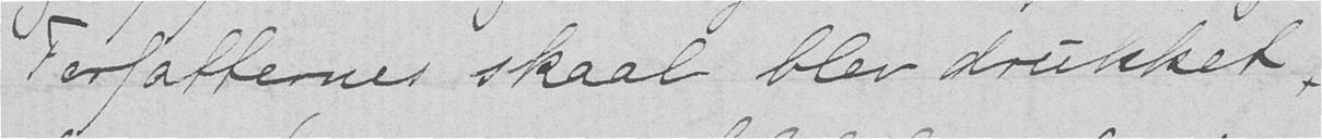Forfatternes skaal blev drukket
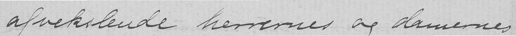afvekslende herrernes og damernes
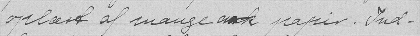oplæst af mange ark papir. Ind-
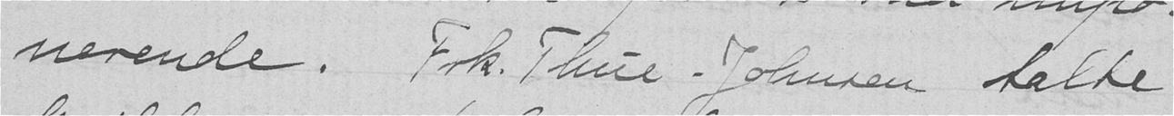nerende. Frk. Thue-Johnsen talte
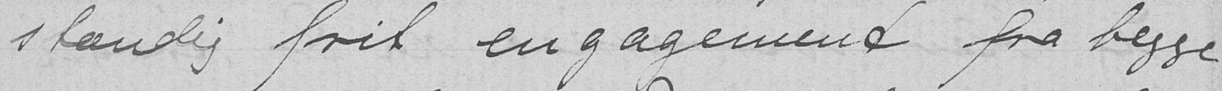stændig frit engagement fra begge
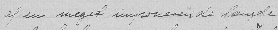af en meget imponerende længde
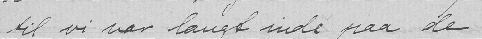til vi var langt inde paa de
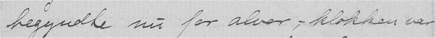begyndte nu for alvor, - klokken var
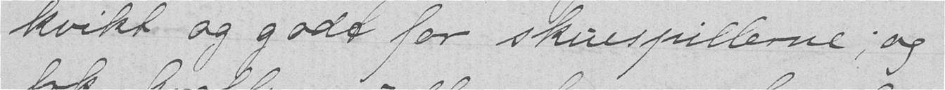kvikt og godt for skuespillerne; og
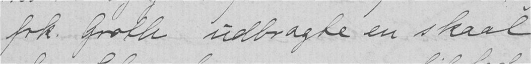frk. Groth udbragte en skaal
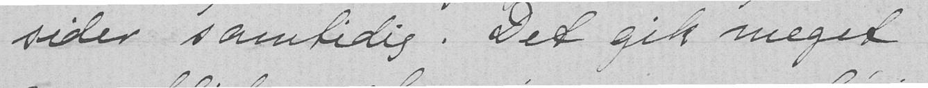sider samtidig. Det gik meget
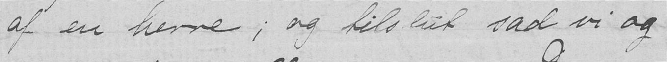af en herre; og tilslut sad vi og
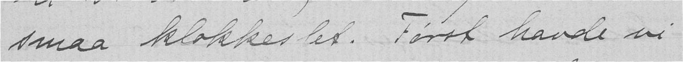smaa klokkeslet. Først havde vi
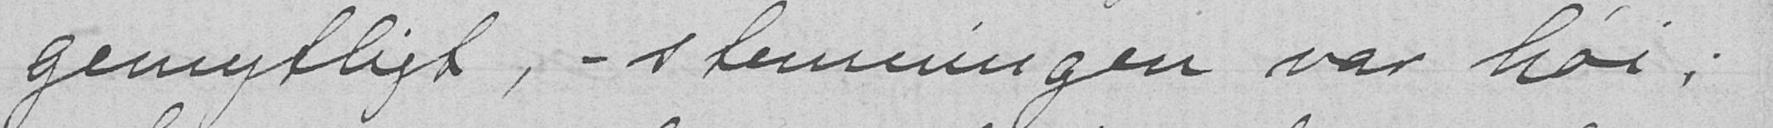gemytligt, - stemningen var høi,
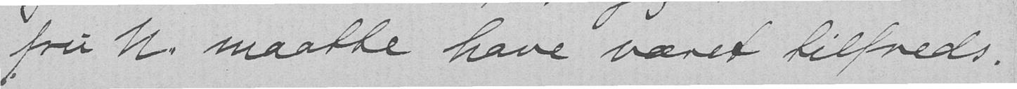fru N. maatte have været tilfreds.
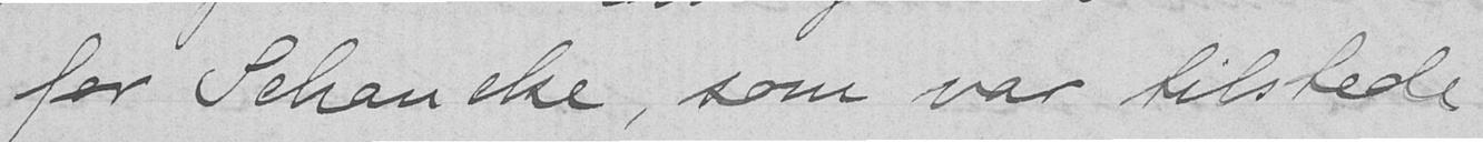for Schancke, som var tilstede
